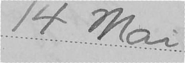14. Mai
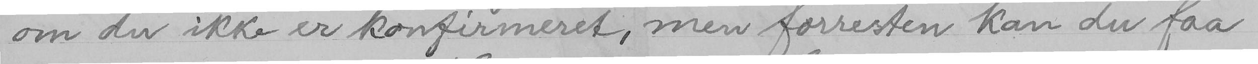om du ikke er konfirmeret, men forresten kan du faa
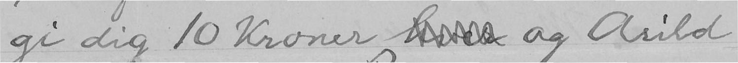gi dig 10 Kroner hver og Arild
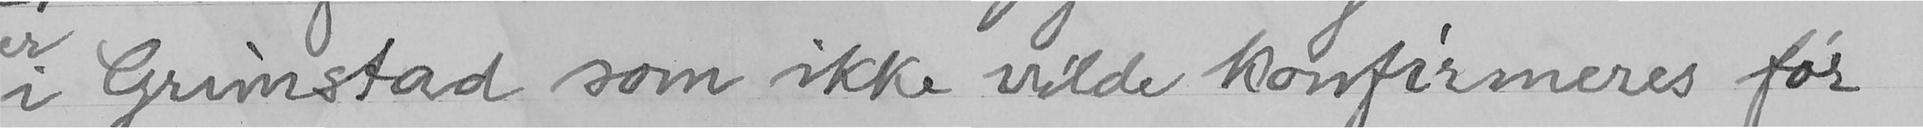i Grimstad som ikke vilde konfirmeres før
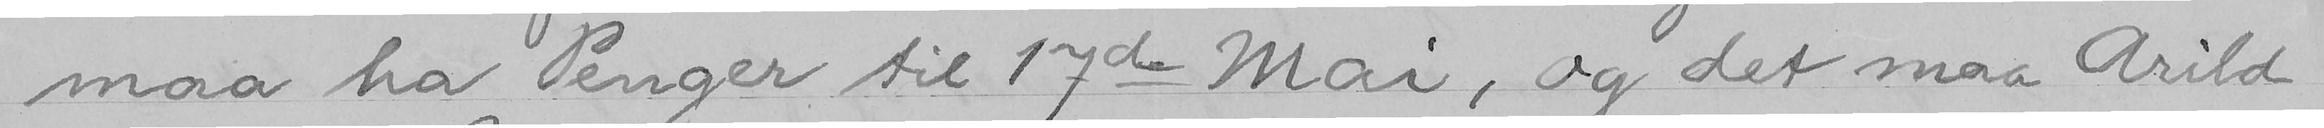maa ha Penger til 17de Mai, og det maa Arild
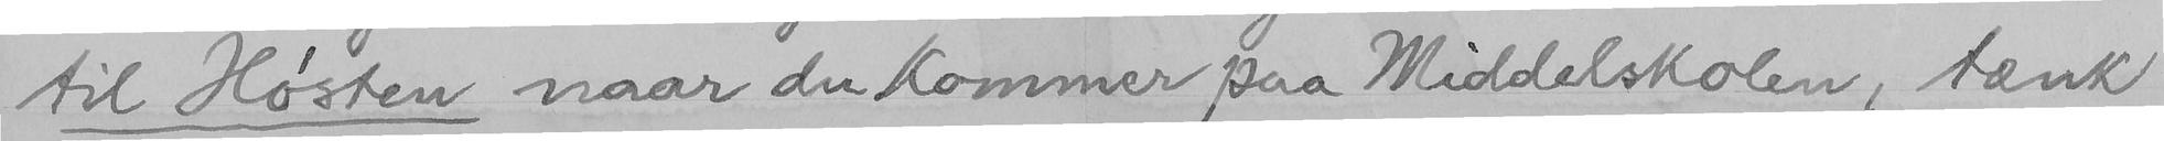til Høsten naar du kommer paa Middelskolen, tænk
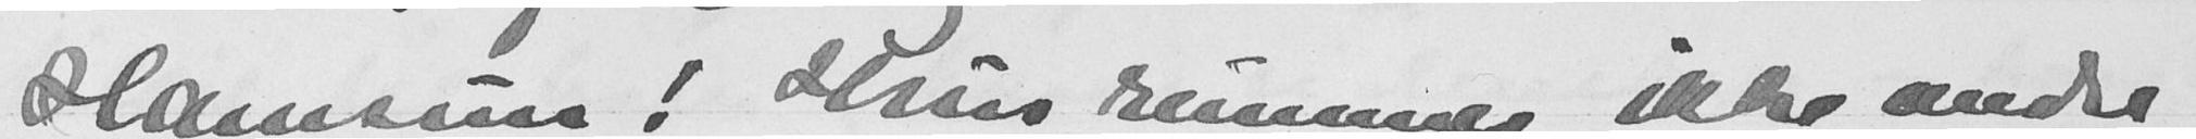Hamsun! Hun rummer ikke andre
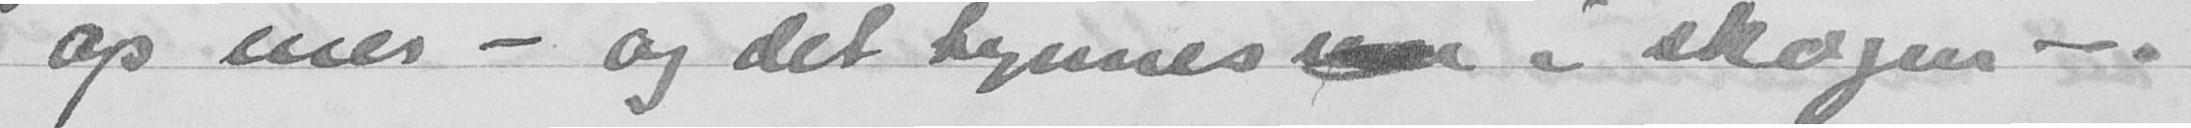op mer - og det tynnes  i skogen.
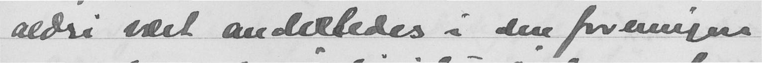aldri vært anderledes i den foreningen
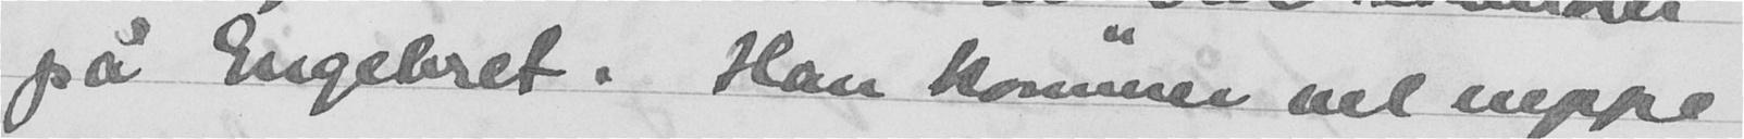på Engebret. Han kommer vel neppe
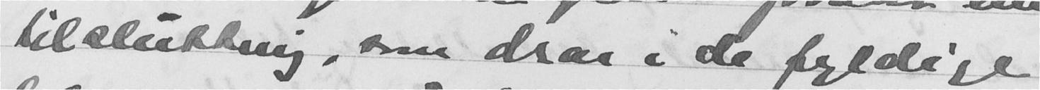tilslutting, som drar i de fyldige
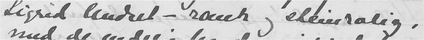Sigrid Undset - rank og stein rolig,
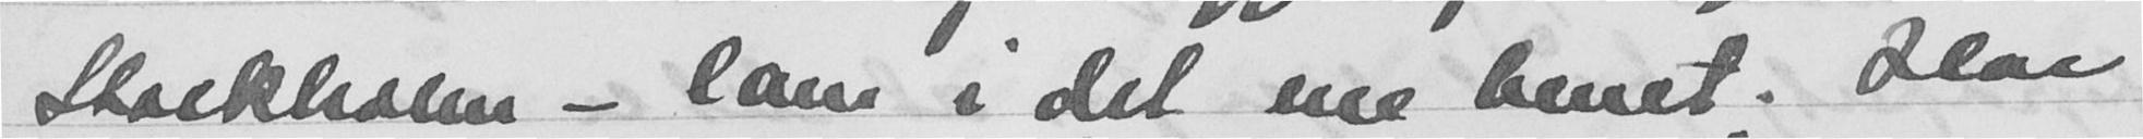Stockholm - lam i det ene benet. Har
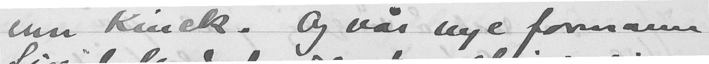enn Kinck. Og vår nye formann
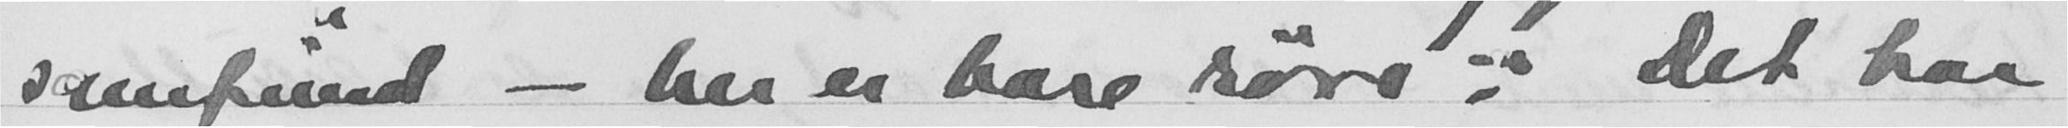samfund - her er bare røre:" Det har
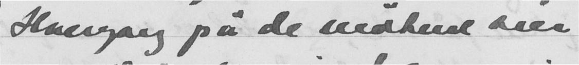Hvergang på de møtene sier
